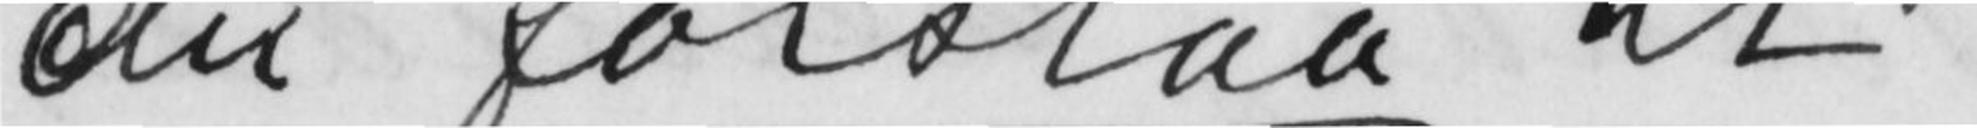der forstaa at
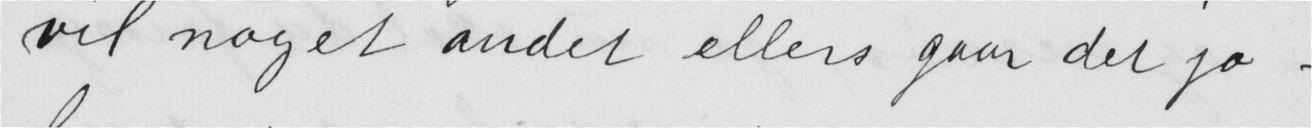vil noget andet ellers gaar det jo
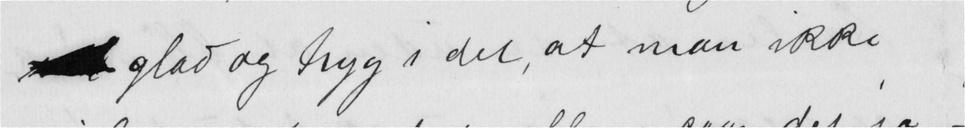m  glad og tryg i det, at man ikke
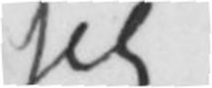jeg
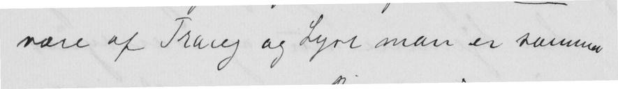være af Trang og Lyre man er sammen
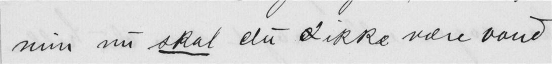min nu skal du d ikke være vond
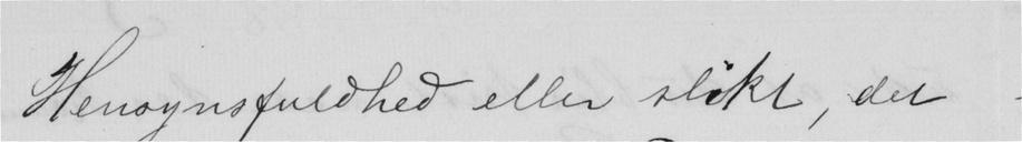Hensynsfuldhed eller slikt, det
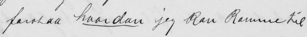forstaa hvordan jeg kan Komme til
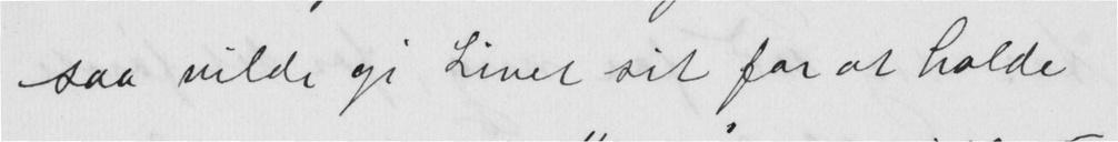saa vilde gi Livet sit for at holde
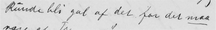kunde bli gal af det for det maa
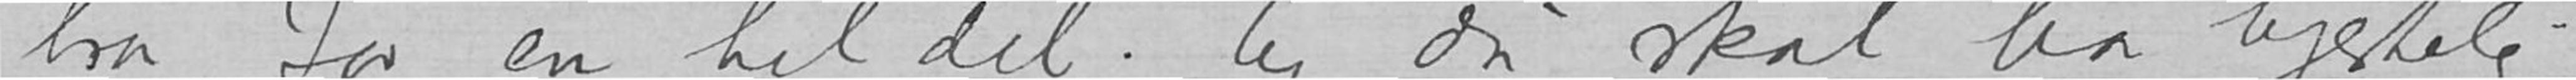bra for en hel del. Og du skal ha hjertelig
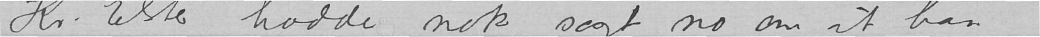Kr. Elster hadde nok sagt no om at han
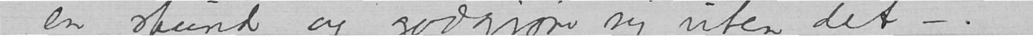en stund og godgjøre sig uten det –.
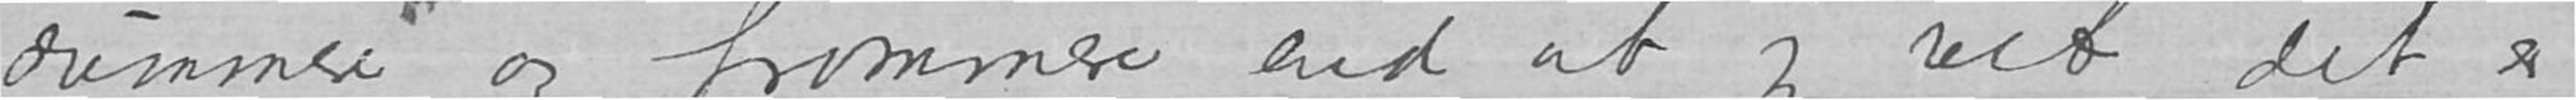dummere og frommere end at jeg vet, det er
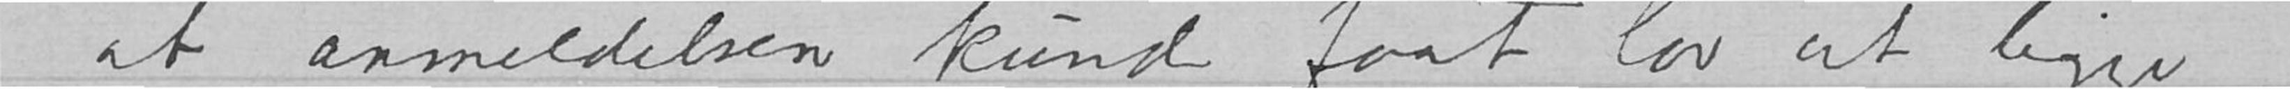at anmeldelsen kunde faat lov at ligge
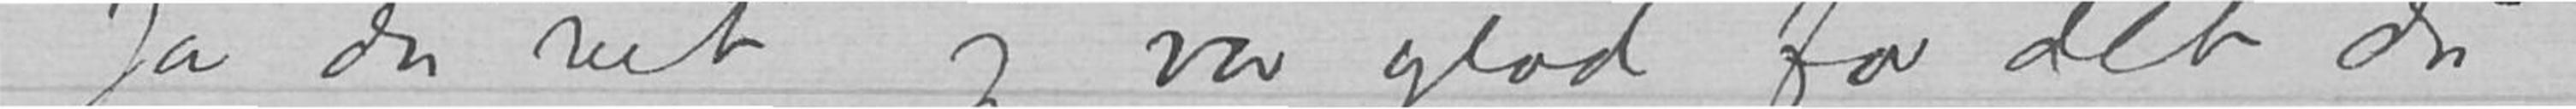Ja du vet, jeg var glad for det du
